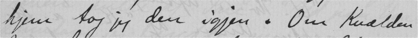hjem tog jeg den igjen. Om Kvælden
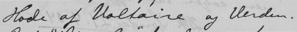Hode af Voltaire og Verden.
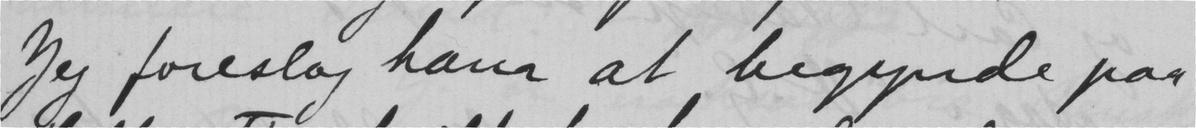Jeg foreslog ham at begynde paa
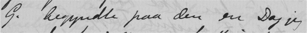G. begyndte paa den en Dag jeg
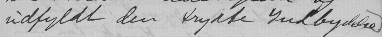udfyldt den trykte Indbydelse.
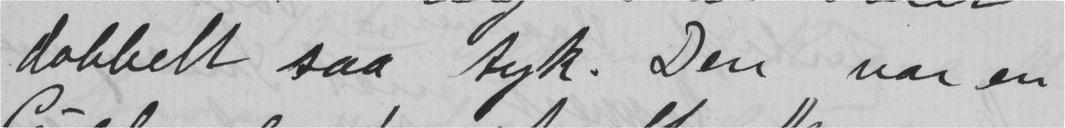dobbelt saa tyk. Den var en
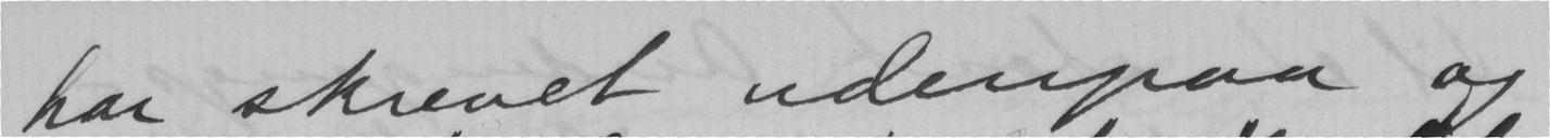har skrevet udenpaa og
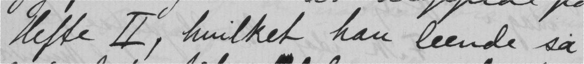Hefte II, hvilket han leende sa
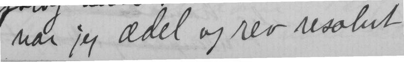var jeg ædel og rev resolut
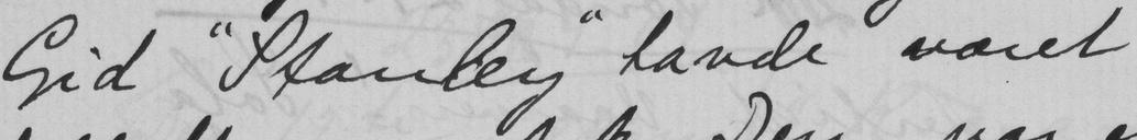Gid "Stanley" havde været
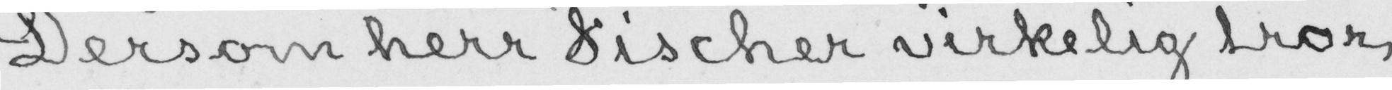Dersom herr Fischer virkelig tror
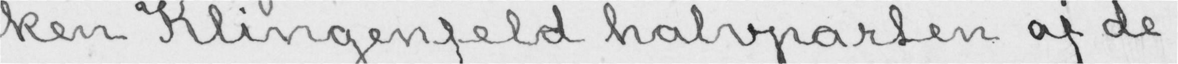ken Klingenfeld halvparten af de
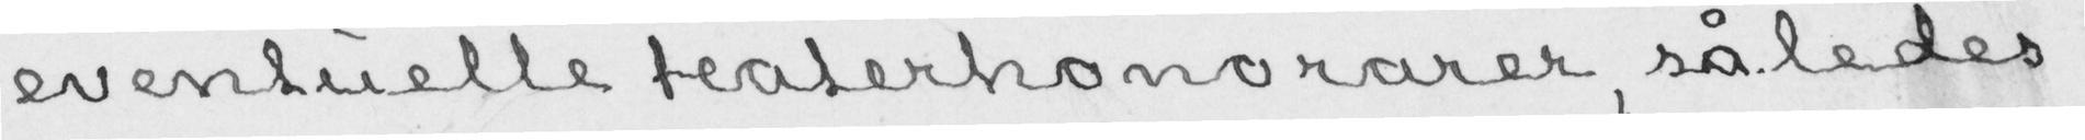eventuelle teaterhonorarer, således
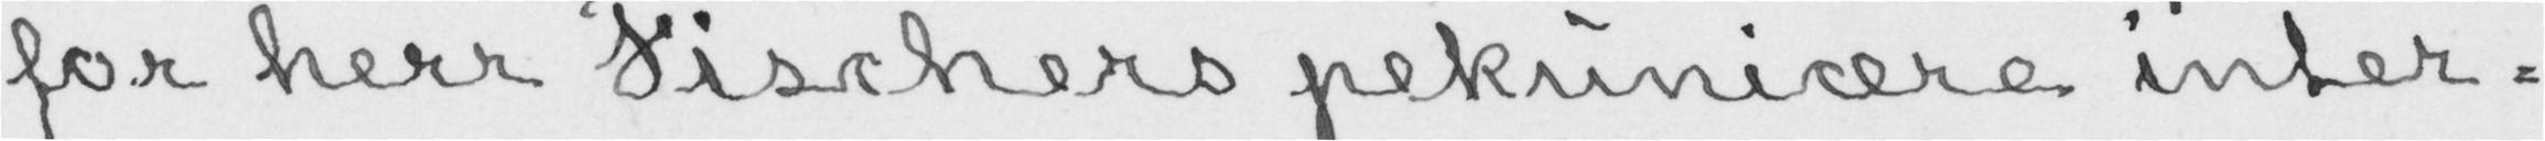for herr Fischers pekuniære inter-
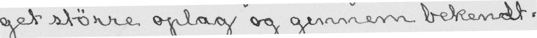get større oplag og gennem bekendt-
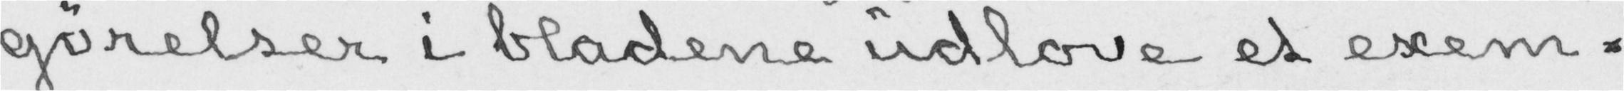gørelser i bladene udlove et exem-
